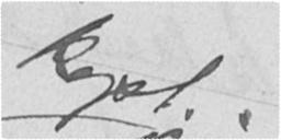kapt.
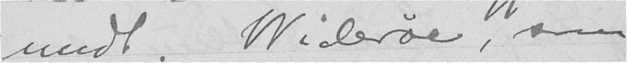undt. Widerøe, som
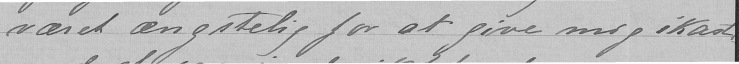været ængstelig for at give mig ikast
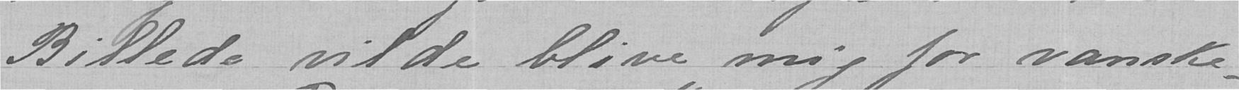Billede, vilde blive mig for vanske-
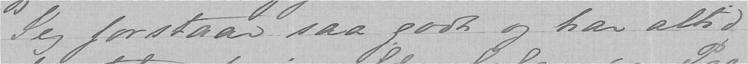Jeg forstaar saa godt og har altid
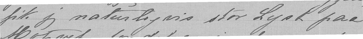fik jeg naturligvis stor Lyst paa
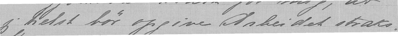jeg helst bør opgive Arbeidet straks.
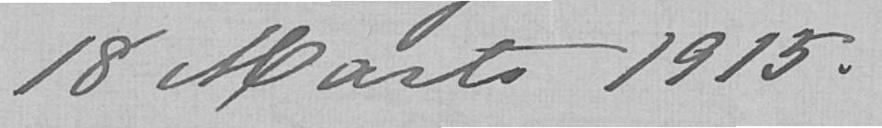18 Marts 1915.
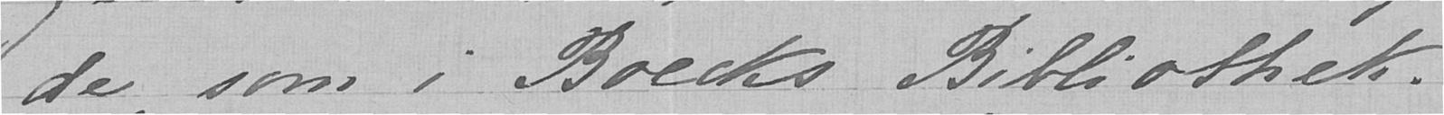de som i Boecks Bibliothek.
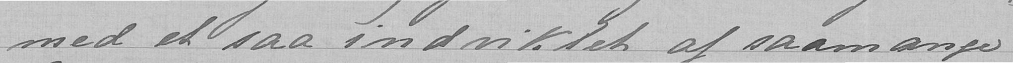med et saa indviklet af saamange
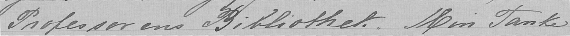Professorens Bibliothek. Min Tanke
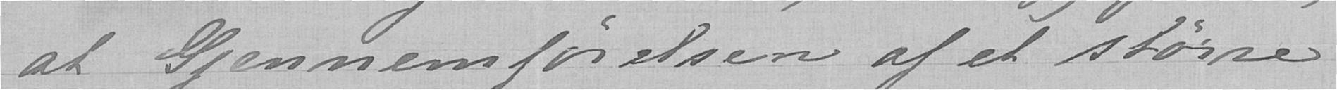at Gjennemførelsen af et større
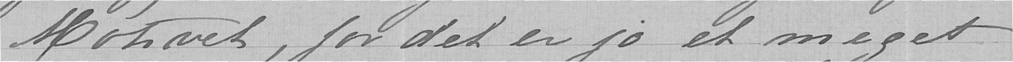Motivet, for det er jo et meget
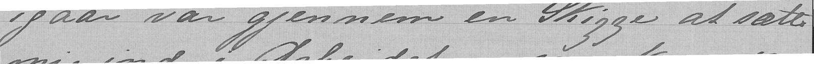igaar var gjennem en Skizze at sætte
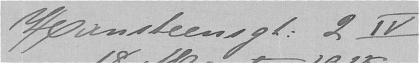Hansteensgt. 2 IV
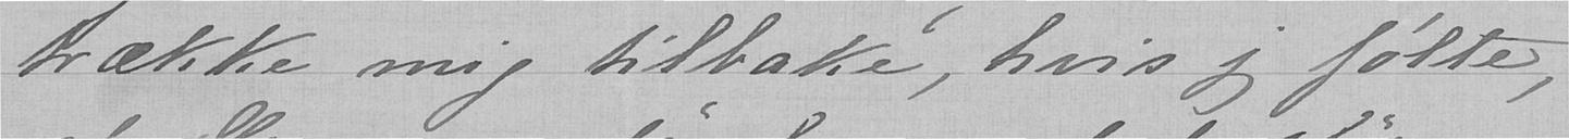trække mig tilbake, hvis jeg følte,
